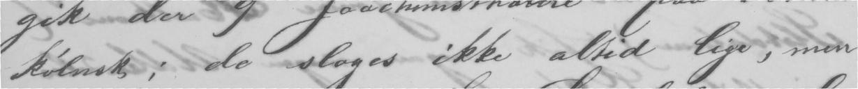kølnsk: de sloges ikke altid lige, men
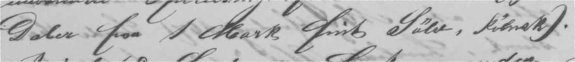Daler paa 1 Mark fint Sølv, kølnsk).
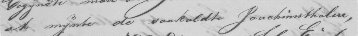at mynte de saakaldte Joachimsthalere,
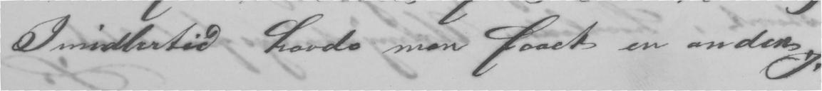Imidlertid havde man faaet en anden
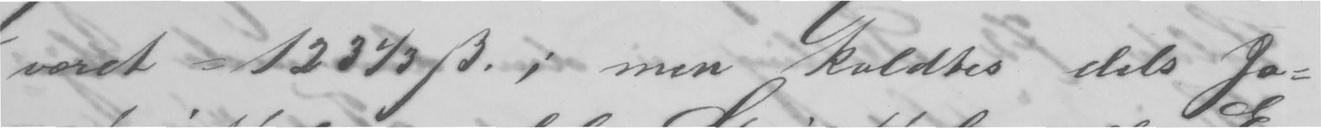været = 12343 ẞ.; men kaldtes dels Jo-
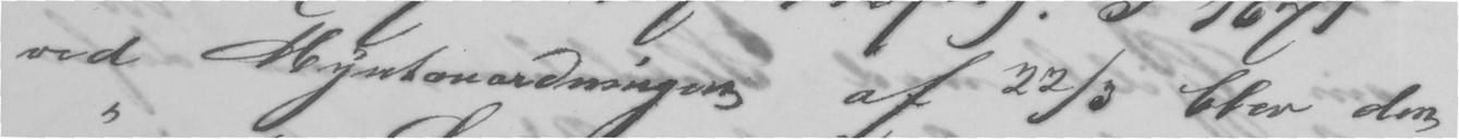ved Myntonordningen af 22/3 blev den
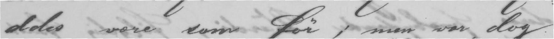deles være som før; men var dog
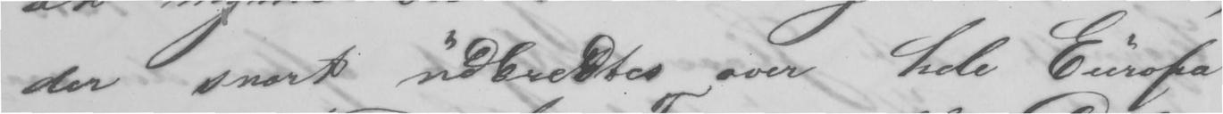der snart udbredtes over hele Europa
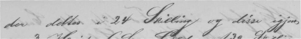der deltes i 24 Skilling og disse igjen
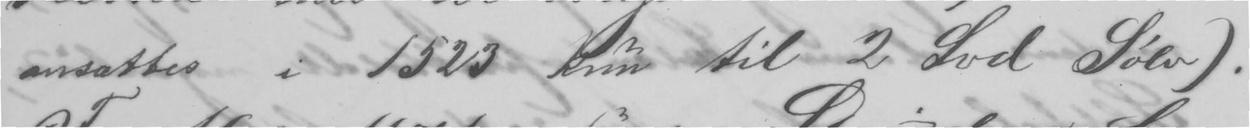omsattes i 1523 kun til 2 Svd Sølv).
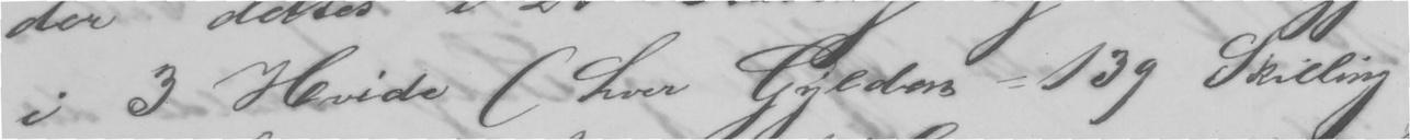i 3 Hvide (hver Gyldens =139 Skilling
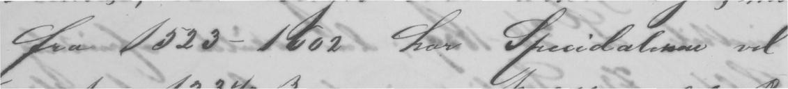fra 1523-1602 har Specidaleren vel
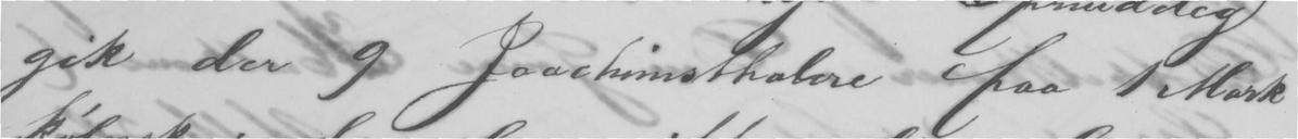gik der 9 Joachimsthaler paa 1 Mark
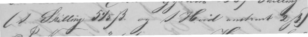(I Skilling 5 ¹⁄₅ ẞ. og 1 Hvid omtrent 2 ẞ)
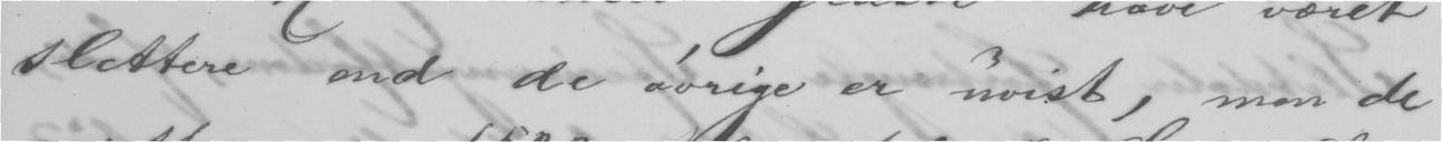slettere end de øvrige er uvist, men de
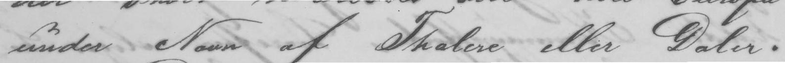under Navn af Thalere eller Daler.
